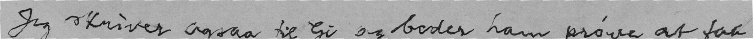Jeg skriver ogsaa til Gi og beder ham prøve at faa
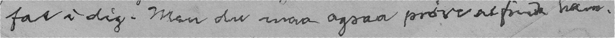fat i dig. Men du maa ogsaa prøve at finde ham.
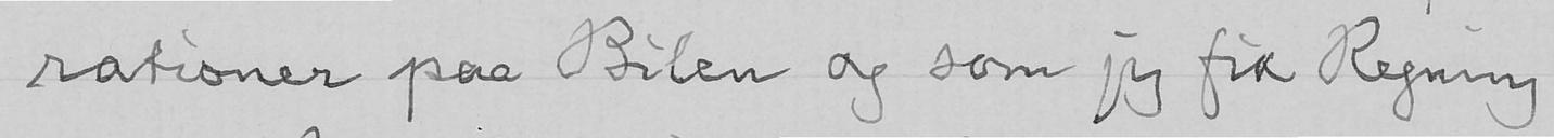rationer paa Bilen og som jeg fik Regning
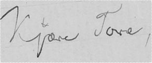Kjære Tore,
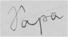Papa
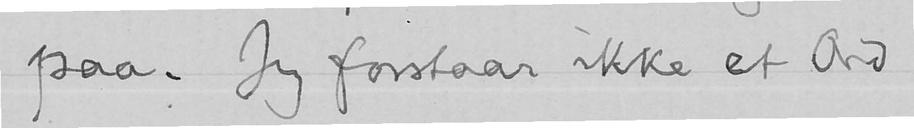paa. Jeg forstaar ikke et Ord
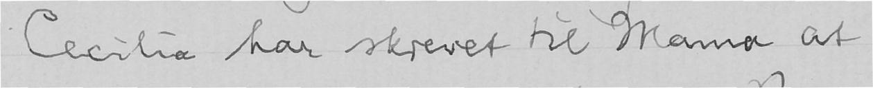Cecilia har skrevet til Mama at
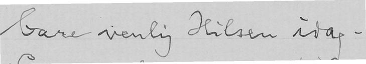bare venlig Hilsen idag.
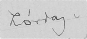Lørdag.
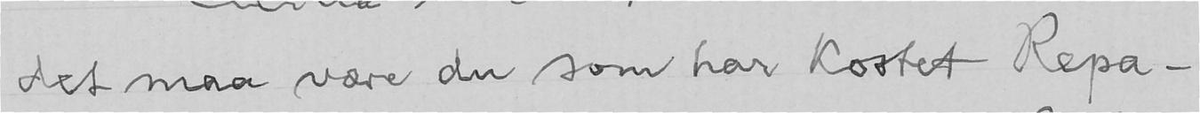det maa være du som har kostet Repa-
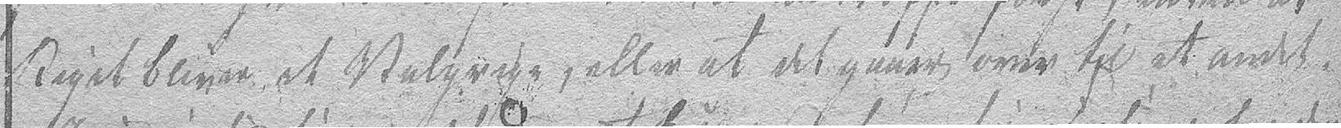Riget bliver et Valgrige, eller at det gaaer over til et andet.
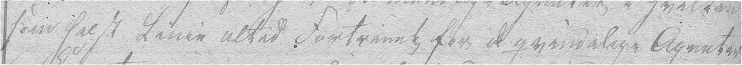som helst Linie altid Fortrinet for de qvindelige Agnater
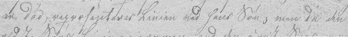er død, repræsenteres Linien ved hans Søn, men da den
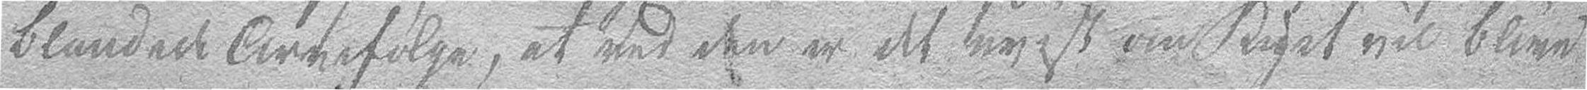blandede Arvefølge, at ved den er det uvist om Riget vil blive
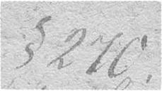§ 270.
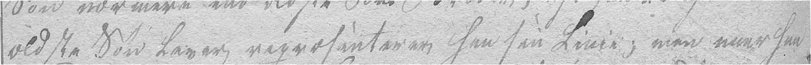ældste Søn lever repræsenterer han sin Linie; men naar han
